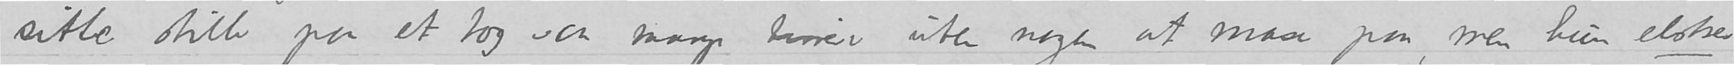sitte stille paa et tog saa mange timer uten nogen at mase paa, men hun elsker
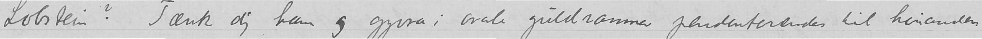Lobstein? Tænk dig han og gyvra i ovale guldrammer prendenterendes til hinanden
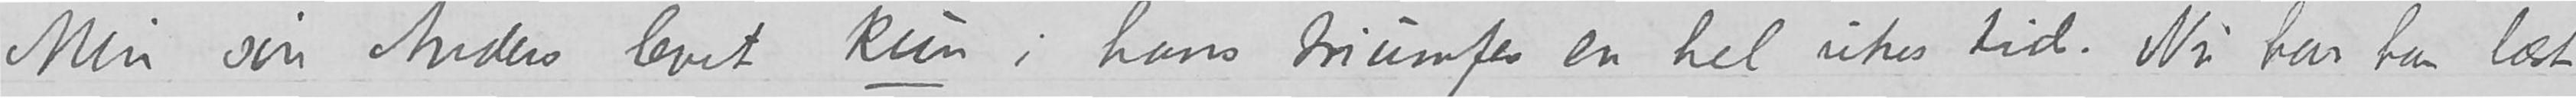Min søn Anders levet kun i hans triumfer en hel ukes tid. Nu har han læst
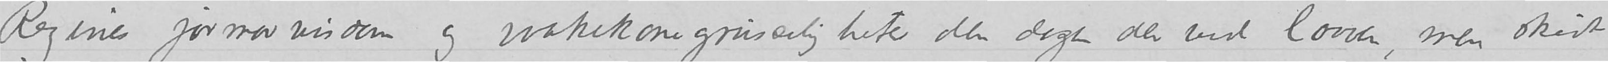Regines jormorvisdom og vaskekonegrusseligheter den dagen der ved lovver/Co? laaven - men skidt
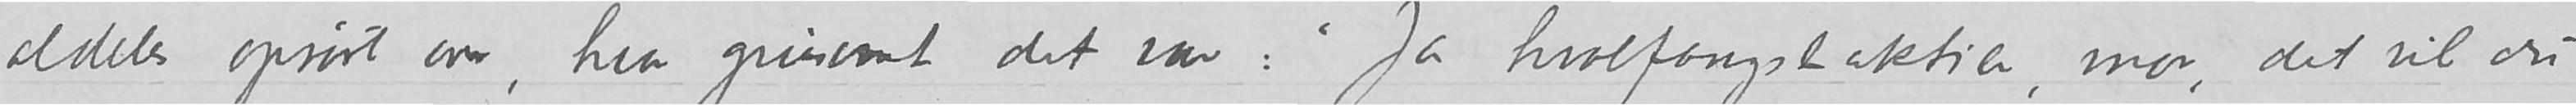aldeles oprørt over, hvor grusomt det var: «Ja hvalfangstlaktier, mor, det vil du
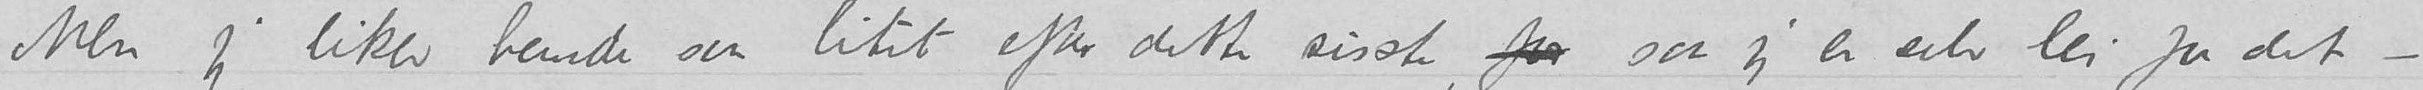Men jeg liker hende saa litet efter dette sisste, for saa jeg er selv lei for det -
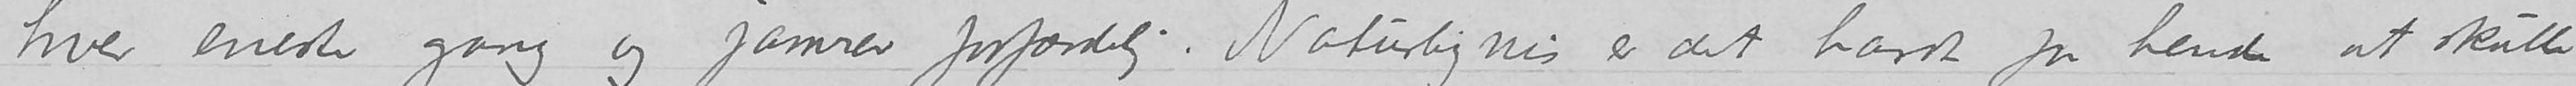hver eneste gang og jamrer forfærdelig. Naturligvis er det hardt for hende at skullde
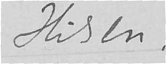Hilsen,
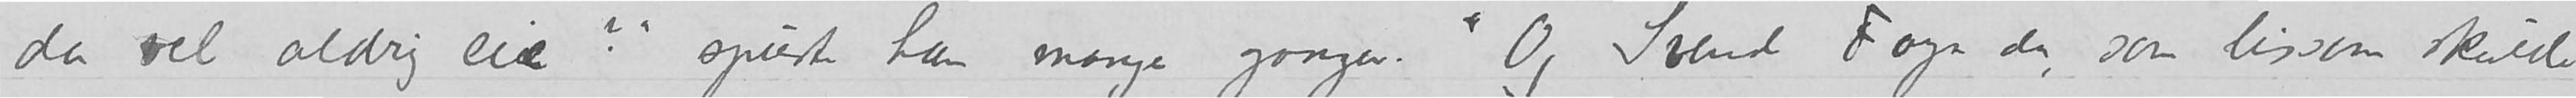da vel aldrig eie?» spurte han mange ganger. «Og Svend Foyn da, som lissom skulde
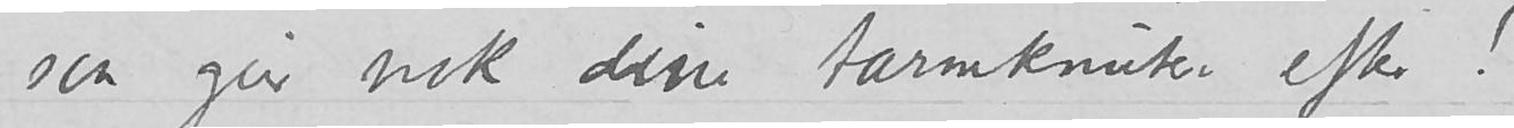saa gir nok dine tarmknuter efter!
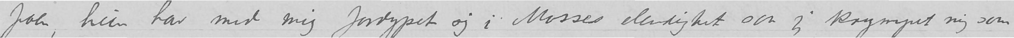faen, hun har med mig fordypet sig i Mosses elendighet saa jeg krympet mig som
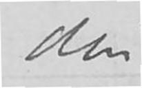din
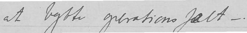at bytte operationsfælt-.
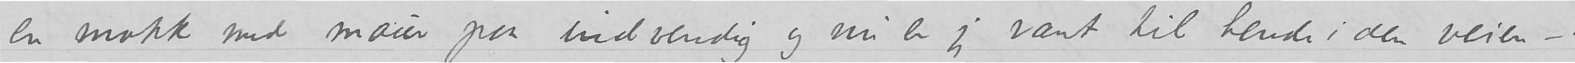en makk med maur paa indvendig og nu er jeg vant til hende i den veien -.
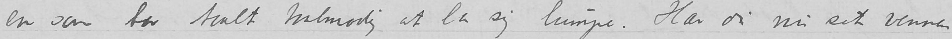en som har taalt taalmodig at la sig lumpe. Har du nu set vennen
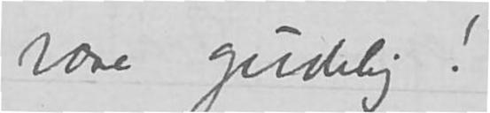være gudelig!»
